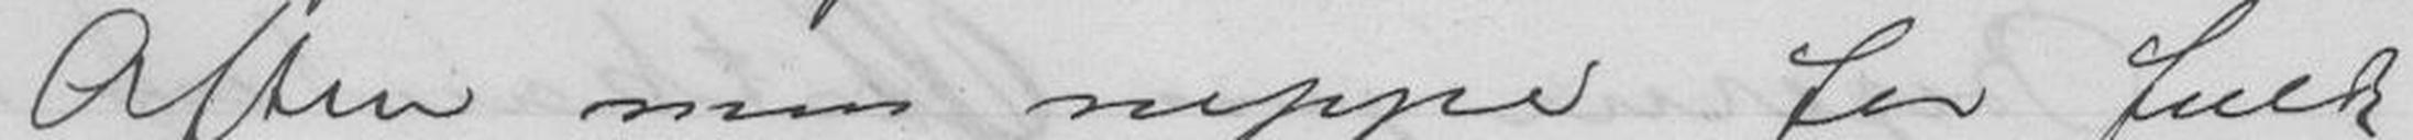aften men neppe for fuldt
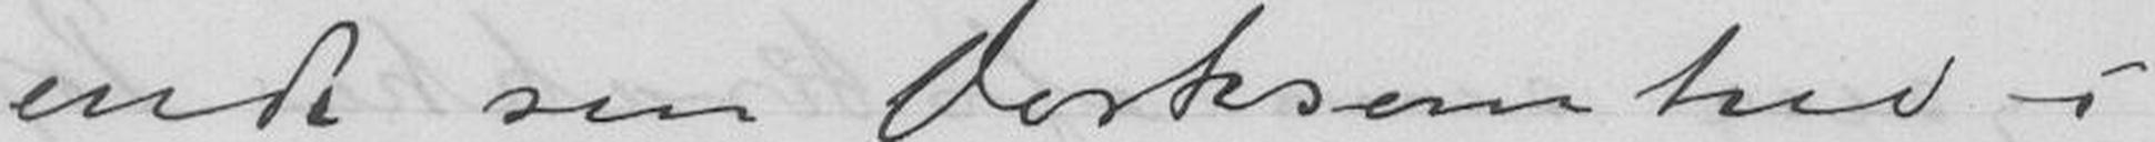endt sin Virksomhed i
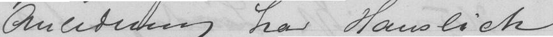Anledning har Hanslich
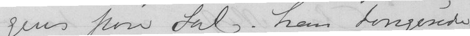gens store Sal han dirigerede
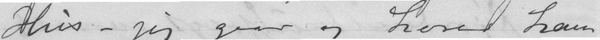Hus - jeg gaar og hører ham
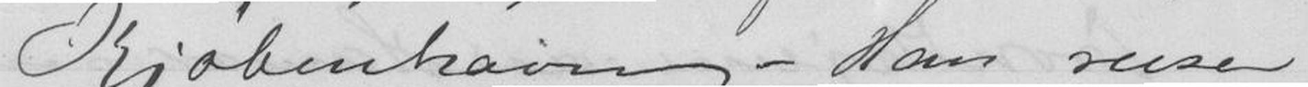Kjøbenhavn - Han reiser
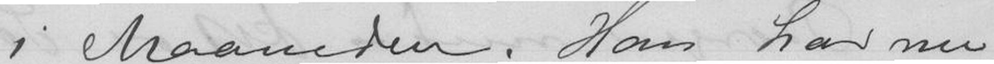i Maaneden. Han har nu
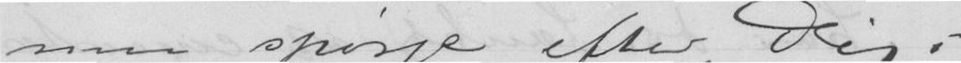maa spørge efter Dig i
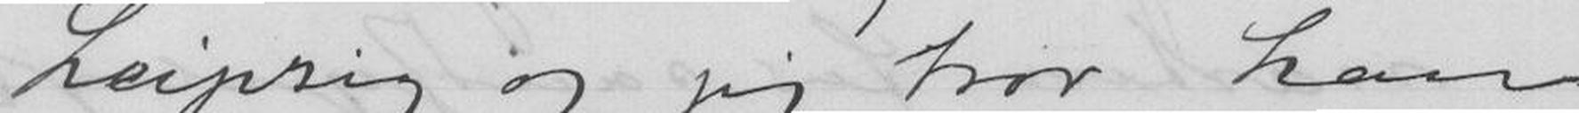Leipzig og jeg tror han
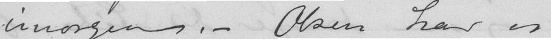imorgen. - Olsen har vi
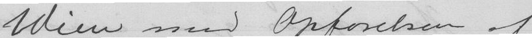Wien med Opførelsen af
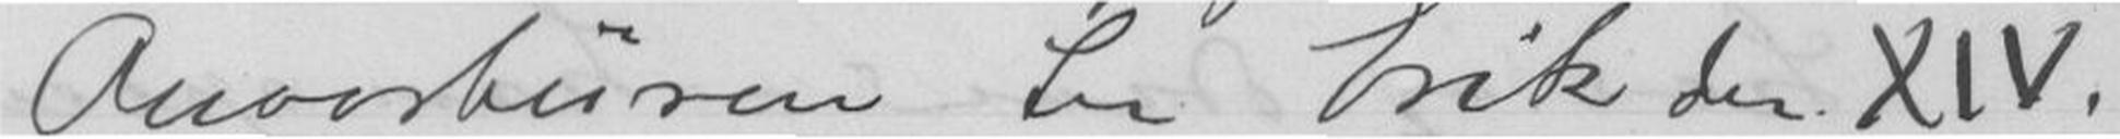Ouverturen til Erik den XIV.
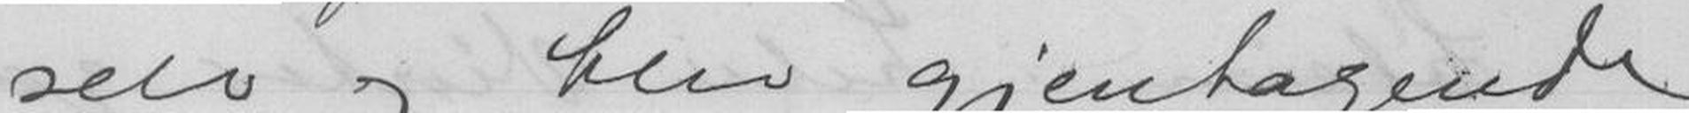selv og blir gjentagende
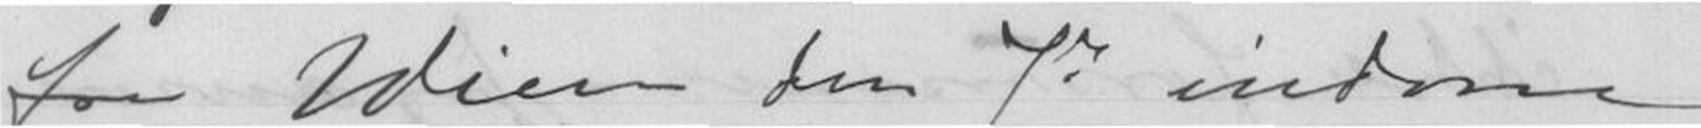fra Wien den 7de indom
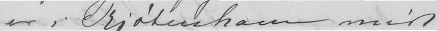er i Kjøbenhavn midt
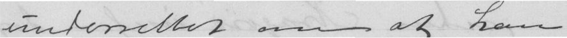underrettet om at han
